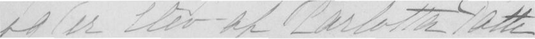og er Elev af Carlotte Patti,
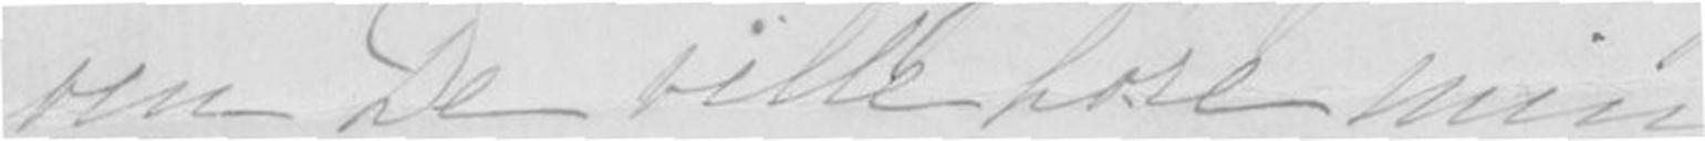om De ville høre min
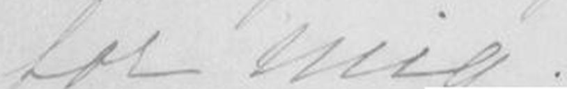for mig.
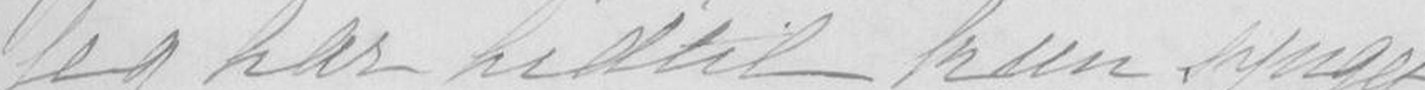jeg har hidtil kun synget
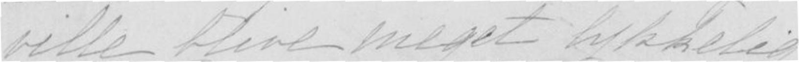ville blive meget lykkelig
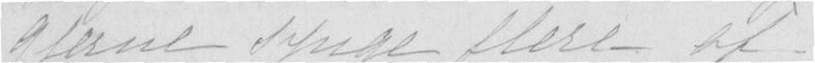gjerne synge flere af
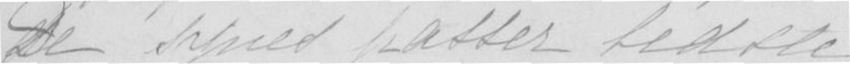De synes passer bedste
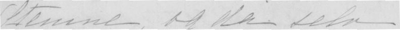stemme, og da selv
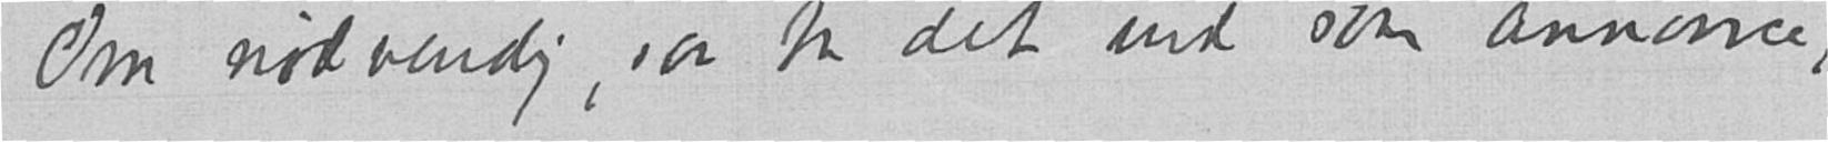Om nødvendig, saa ta det ind som annonce,
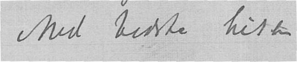Med bedste hilsen
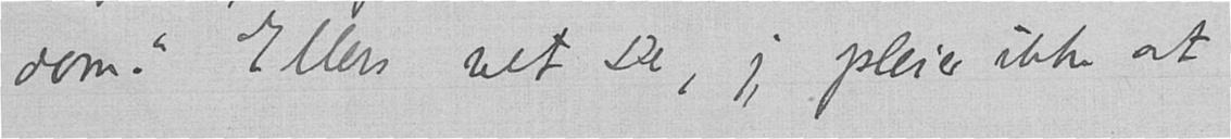dom." Ellers vet De, jeg pleier ikke at
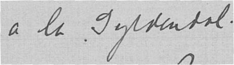a la Gyldendal.
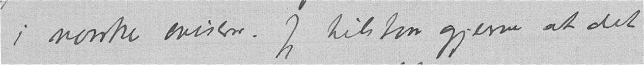i norske aviser. Jeg tilstaar gjerne at det
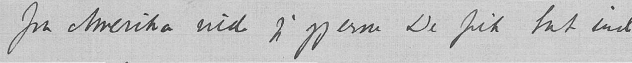fra Amerika vilde jeg gjerne De fik tat ind
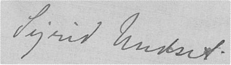Sigrid Undset.
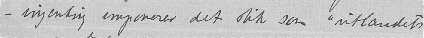- ingenting imponerer det slik som "utlandets
